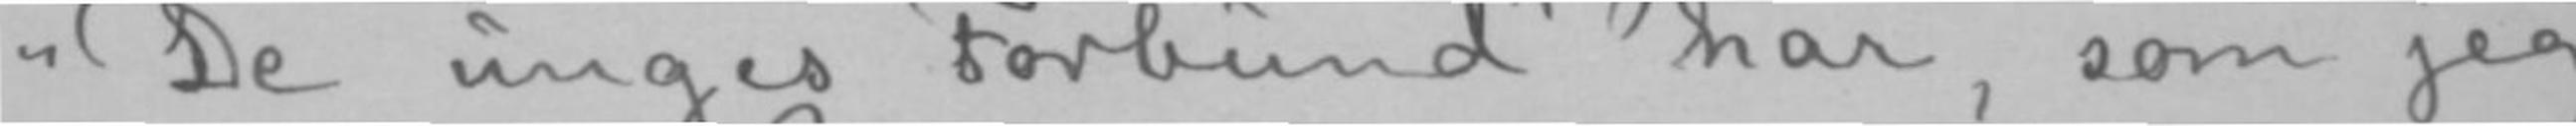«De unges Forbund» har, som jeg
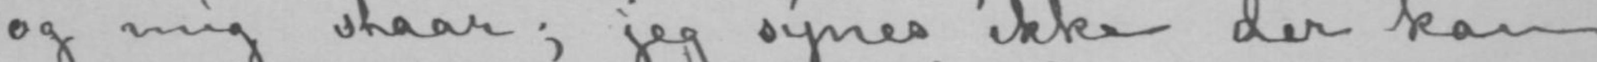og mig staar; jeg synes ikke der kan
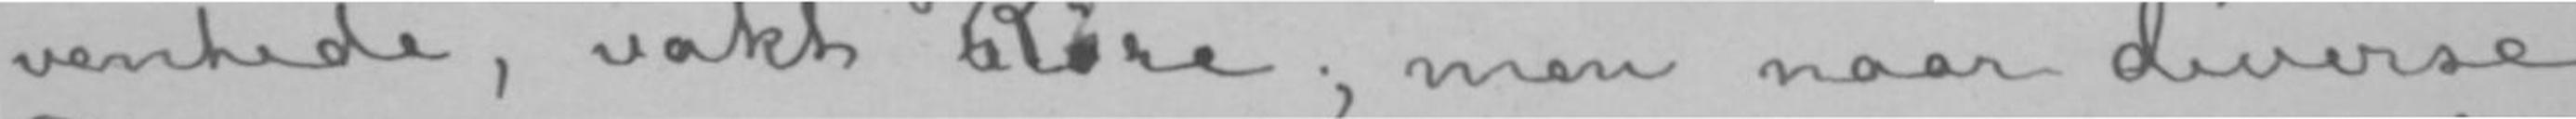ventede, vakt Røre; men naar diverse
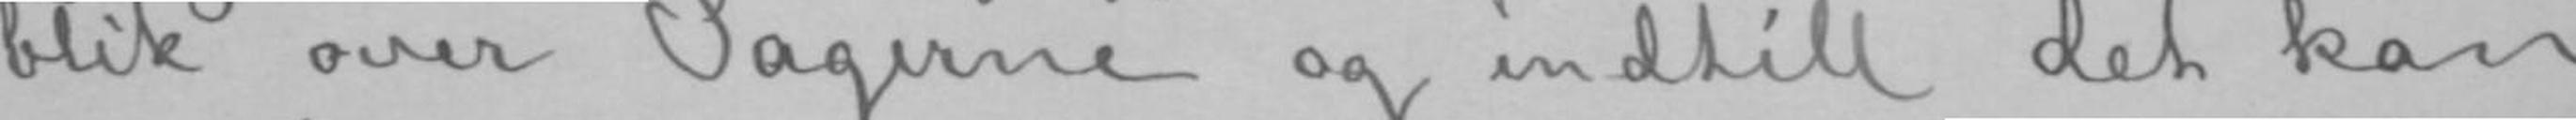blik over Sagerne og indtill det kan
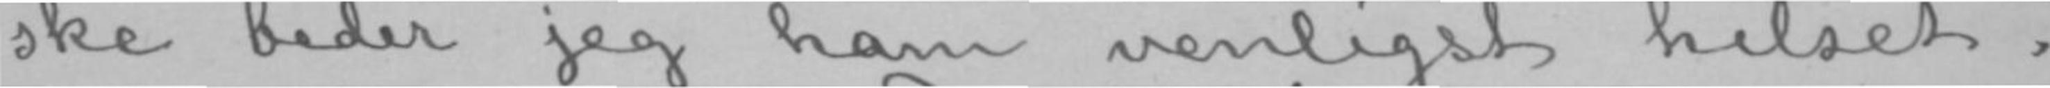ske beder jeg ham venligst hilset.
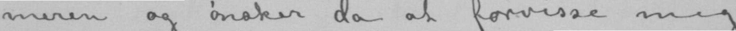meren og ønsker da at forvisse mig
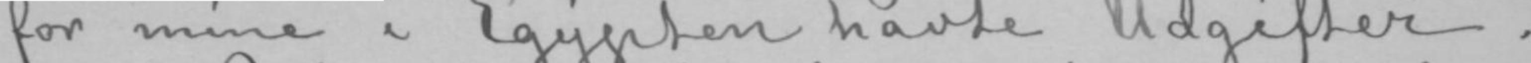for mine i Egypten havte Udgifter.
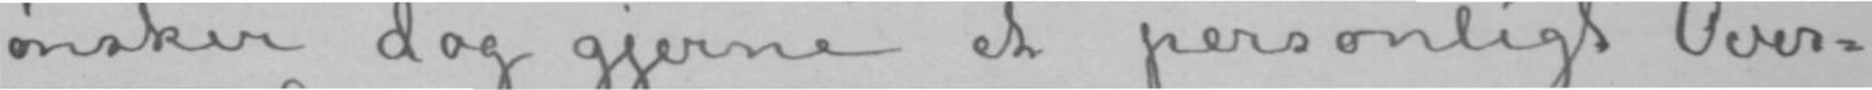jeg ønsker dog gjerne et personligt Over-
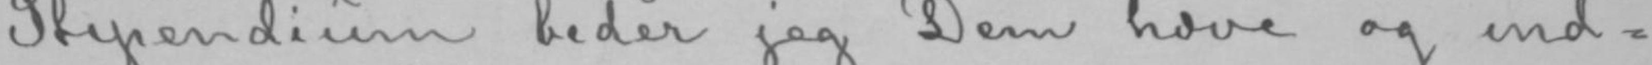Stipendium beder jeg Dem hæve og ind-
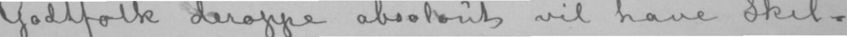Godtfolk deroppe absolout vil have Skil-
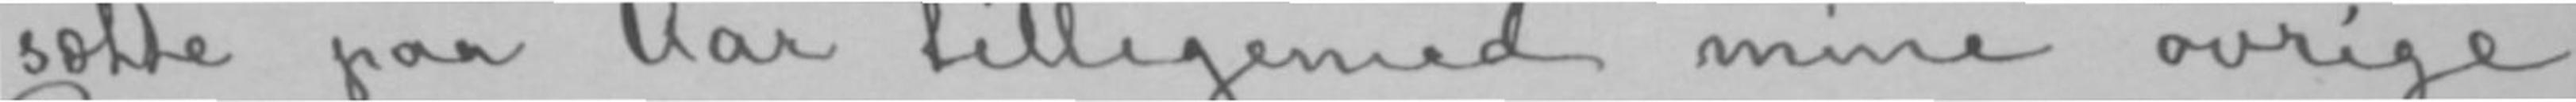sætte paa Aar tilligemed mine øvrige
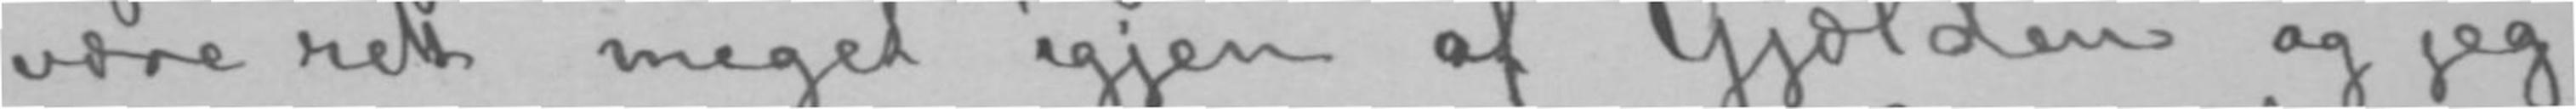være rett meget igjen af Gjælden og jeg
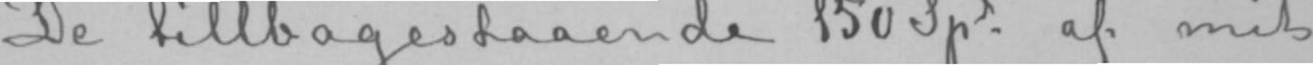De tillbagestaaende 150 Spd af mit
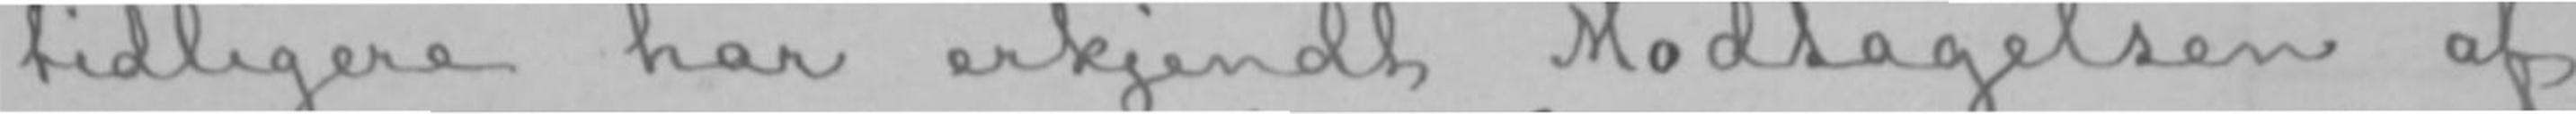tidligere har erkjendt Modtagelsen af
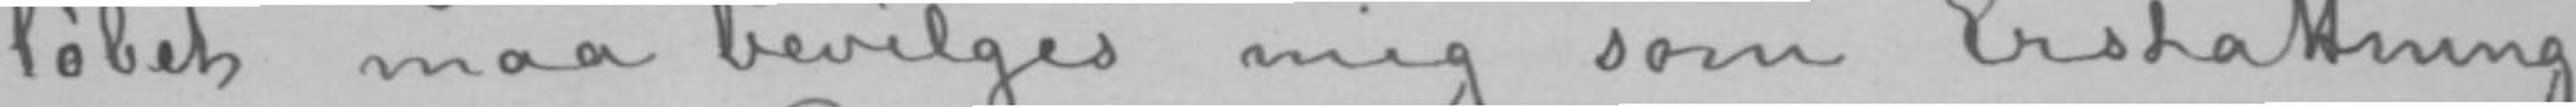løbet maa bevilges mig som Erstattning
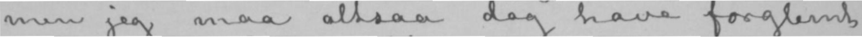men jeg maa altsaa dog have forglemt
